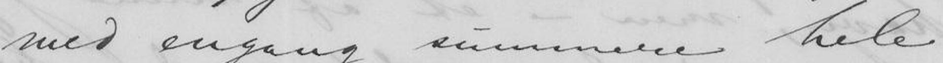med engang summere hele
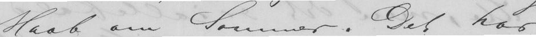Haab om Sommer. Det har
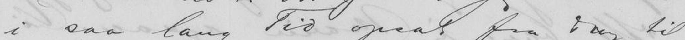i saa lang Tid opsat fra Dag til
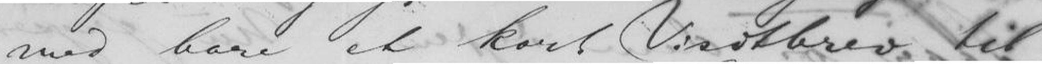med bare et kort Visitbrev til
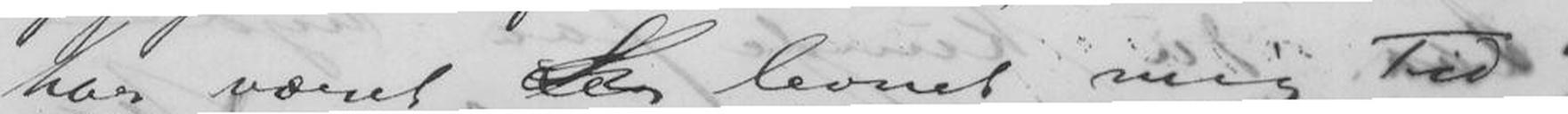har været levnet mig Tid og
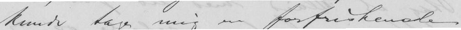kunde tage mig forfriskende
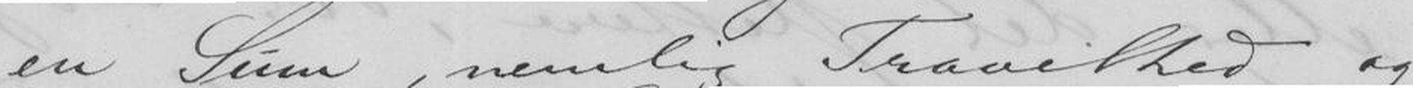en Sum, nemlig Travelhed og
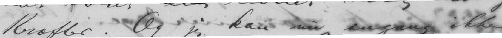Kræfter. Og jeg kan nu engang ikke
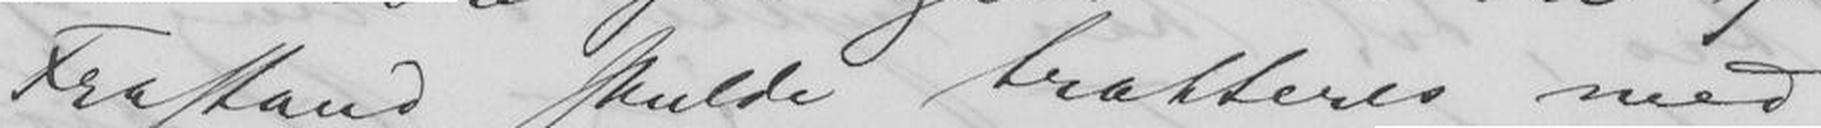Frastand skulde trakteres med
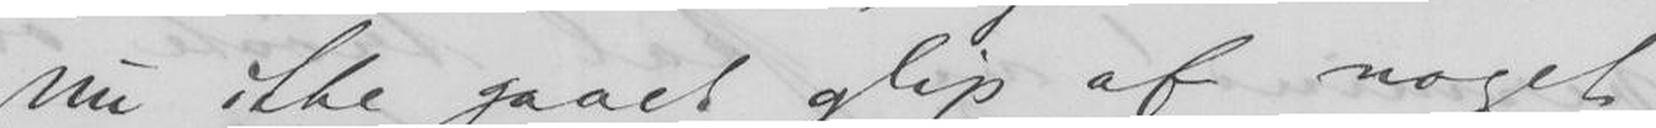nu ikke gaaet glip af noget
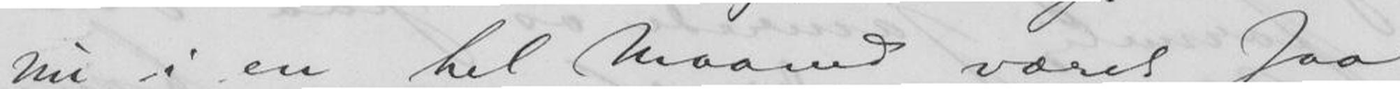nu i en hel Maaned været saa
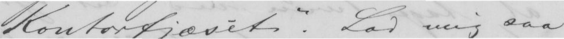"Kontorfjæset". Lad mig saa
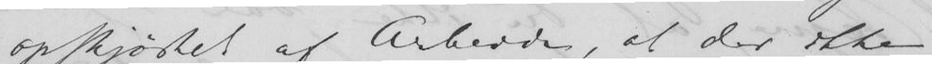opskjørtet af Arbeide, at der ikke
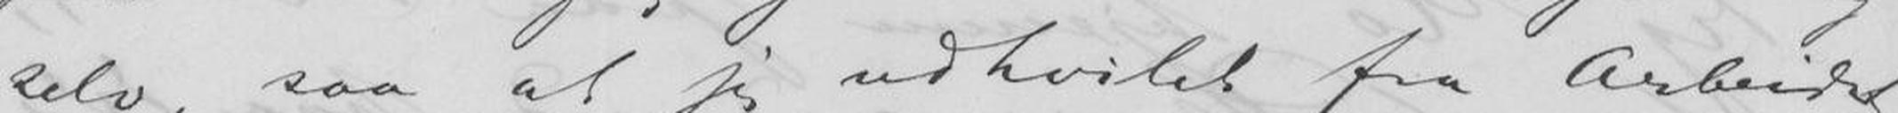selv, saa at jeg udhvilet fra Arbeidet
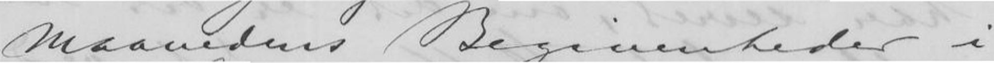Maanedens Begivenheder i
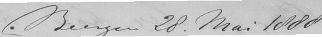Bergen 28. Mai 1888
